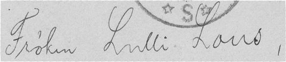Frøken Lulli Lous,
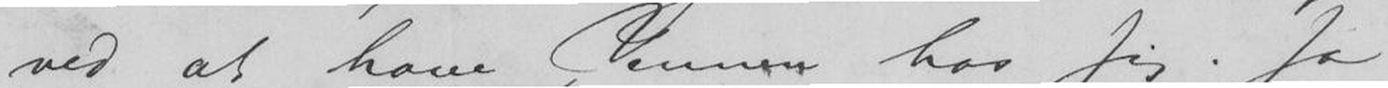ved at have Venner hos sig. Ja
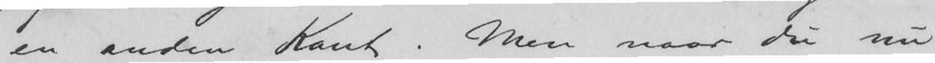en anden Kant. Men naar Du nu
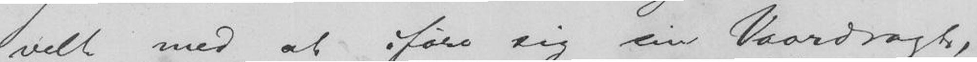velt med at iføre sig sin Vaardragt,
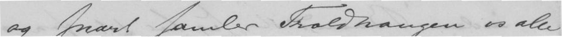og snart samler Troldhaugen os alle
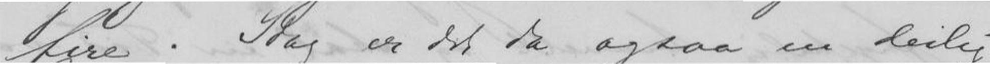fire. Idag er det da ogsaa en deilig
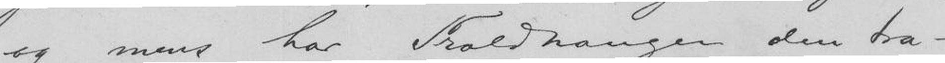og mens har Troldhaugen den tra-
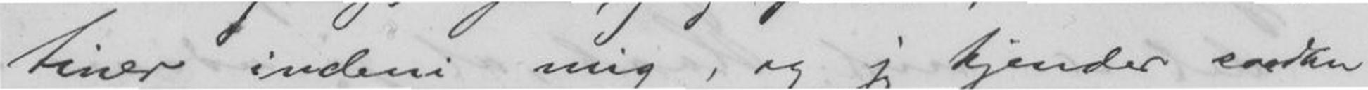tiner indeni mig, og jeg kjender saadan
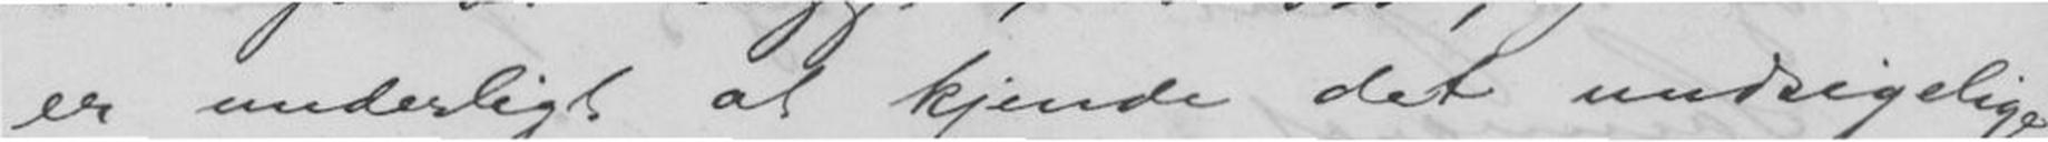er underligt at kjende det uudsigelige,
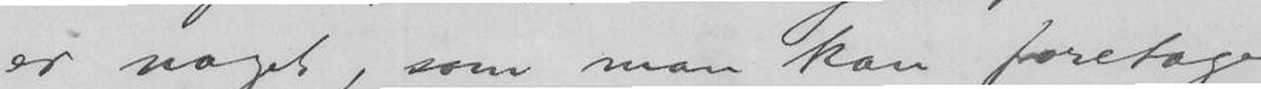er noget, som man kan foretage
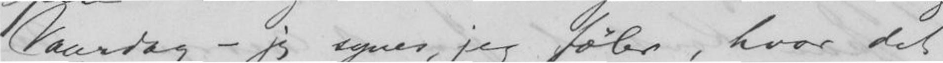Vaardag - jeg synes, jeg føler, hvor det
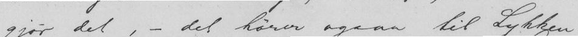gjør det, - det hører ogsaa til Lykken
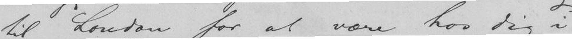til London for at være hos Dig i
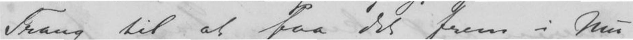Trang til at faa det fram i Mu-
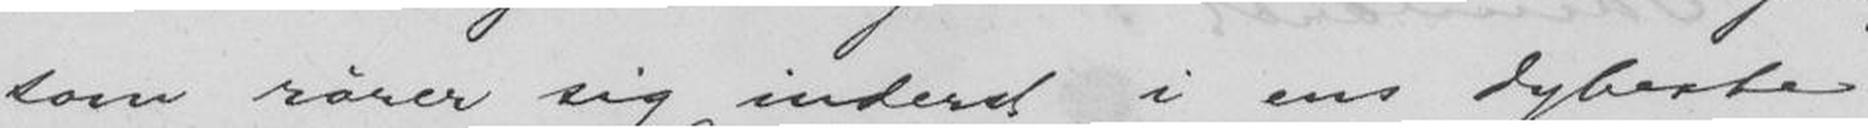som rører sig inderst i ens dybeste
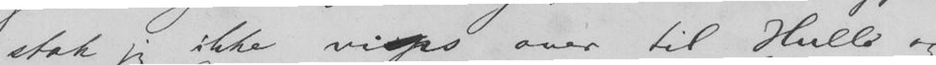stak jeg ikke vips over til Hull og
